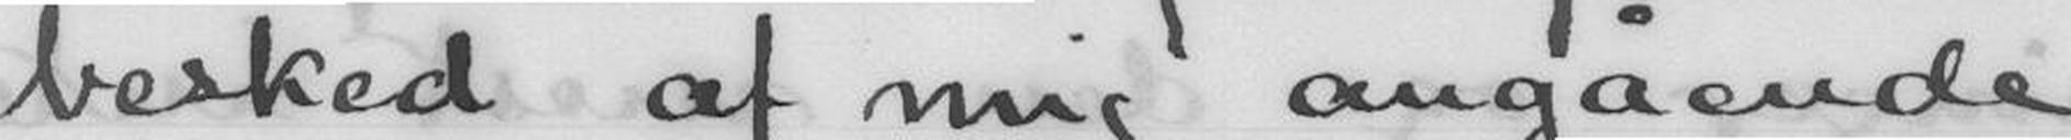besked af mig angående
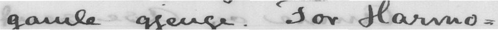gamle gjenge. For Harmo-
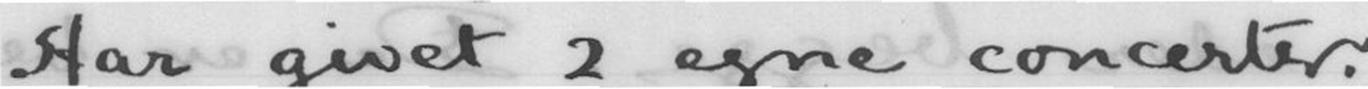Har givet 2 egne concerter.
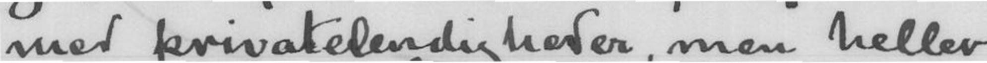med privatelendigheder, men heller
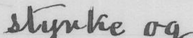styrke og
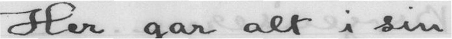Her gar alt i sin
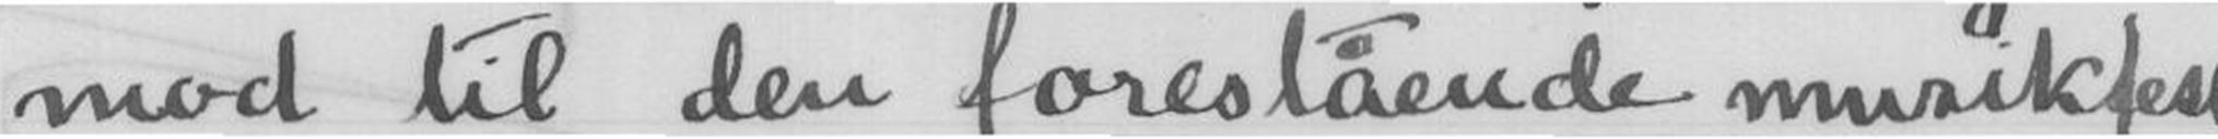mod til den forestående musikfest
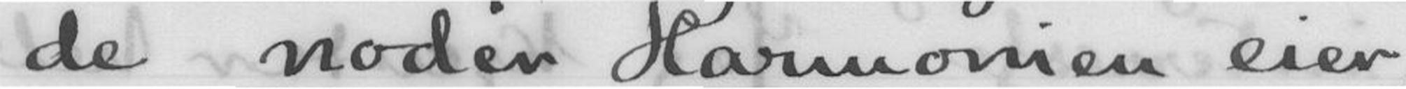de noder Harmonien eier,
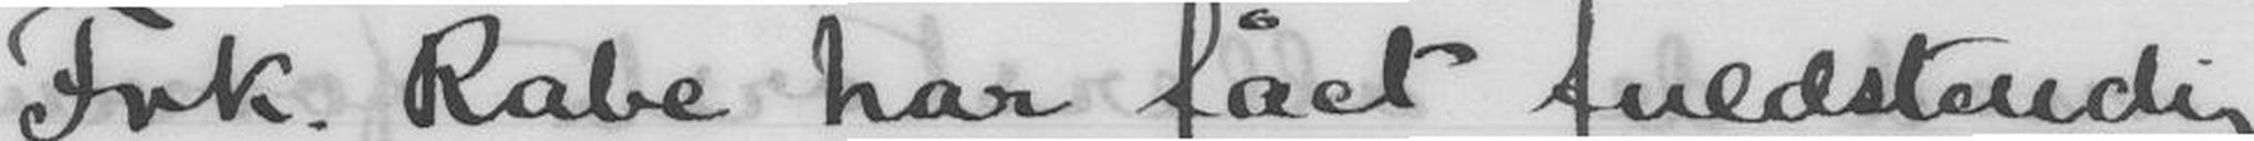Frk. Rabe har fået fuldstendig
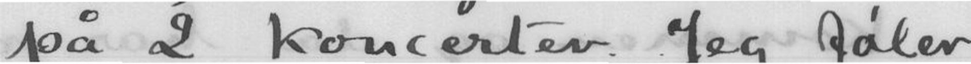på 2 koncerter. Jeg føler
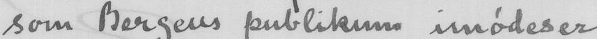som Bergens publikum imødeser
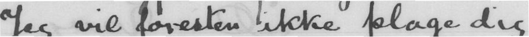Jeg vil foresten ikke plage dig
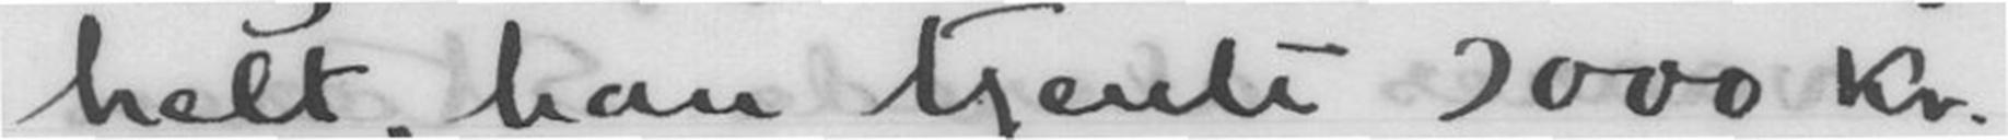helt, han tjente 1000 kr.
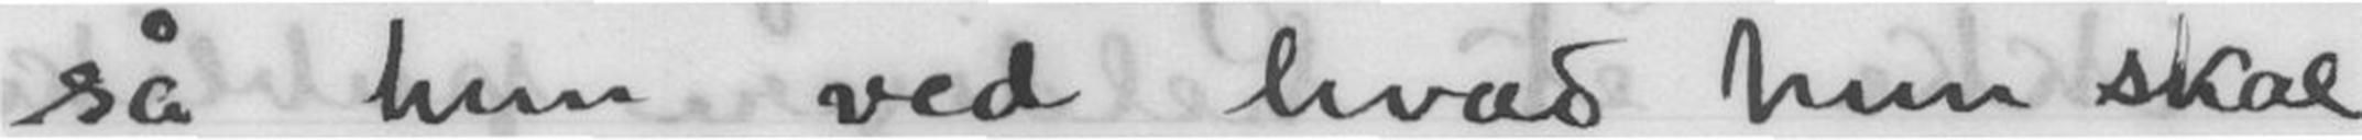så hun ved hvad hun skal
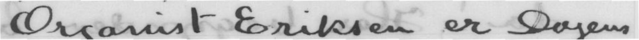Organist Eriksen er Dagens
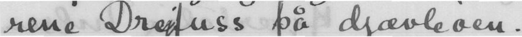rene Dreyfuss på djævleøen.
